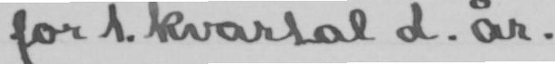for 1. kvartal d. år.
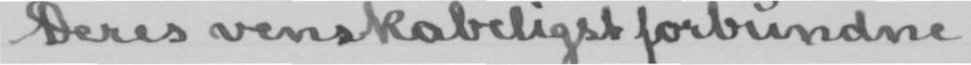Deres venskabeligst forbundne
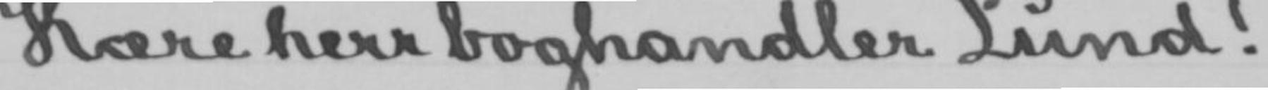Kære herr boghandler Lund!
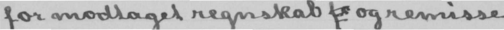for modtaget regnskab fo og remisse
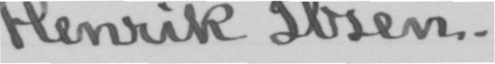Henrik Ibsen.
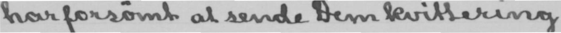har forsømt at sende Dem kvittering
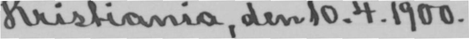Kristiania, den 10.4.1900.
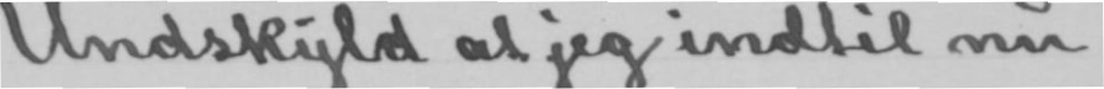Undskyld at jeg indtil nu
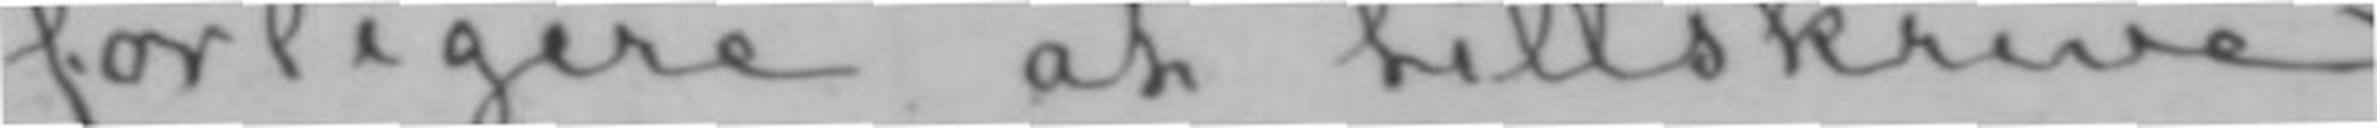førligere at tillskrive
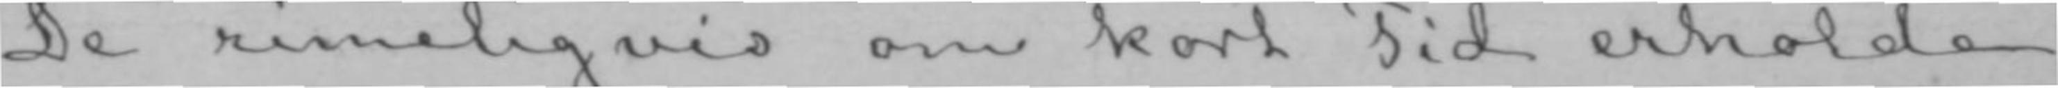De rimeligvis om kort Tid erholde
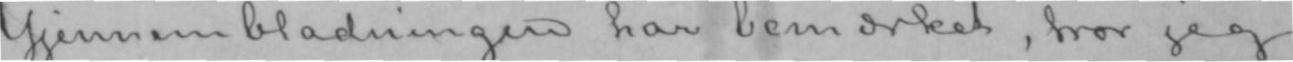Gjennembladningen har bemærket, tror jeg
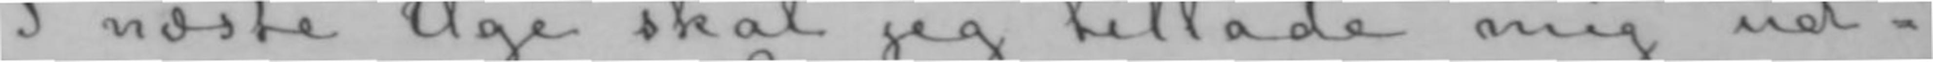I næste Uge skal jeg tillade mig ud-
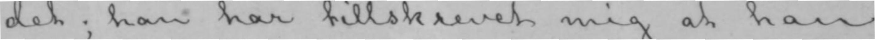det; han har tillskrevet mig at han
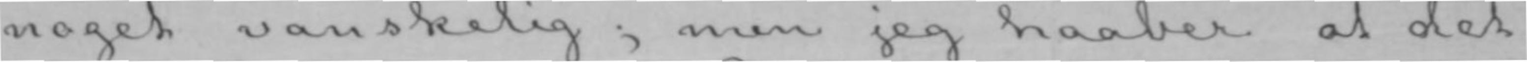noget vanskelig; men jeg haaber at det
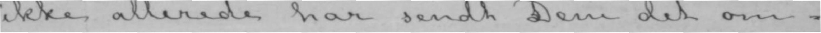ikke allerede har sendt Dem det om-
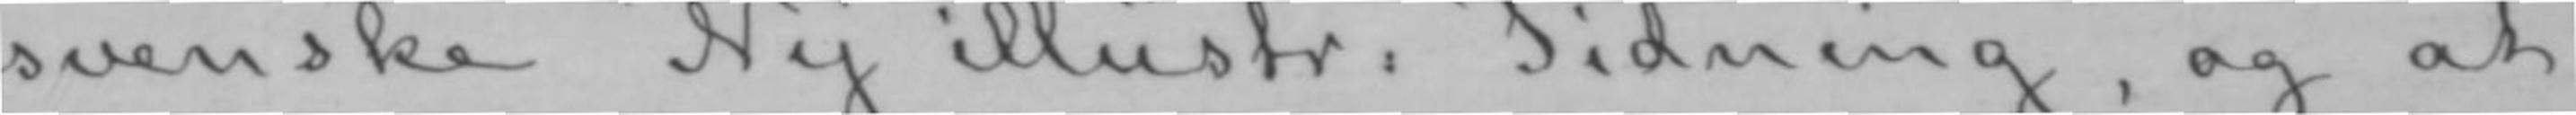svenske «Ny illustr: Tidning», og at
